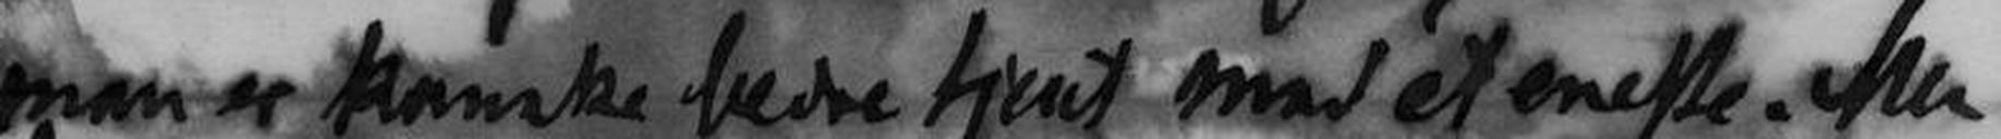man er kanske bedre tjent med et eneste. Men
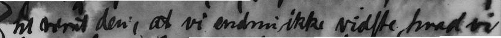til været den, at vi endnu ikke vidste, hvad vi
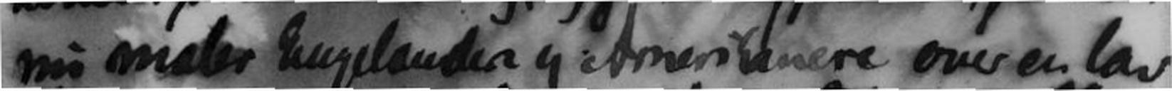nu maler Engelændere og Amerikanere over en lav
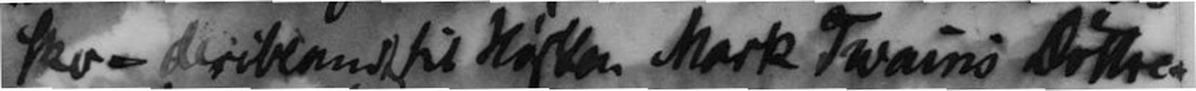Sko - deriblandt til Høsten. Mark Twains Døttre.
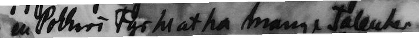en Pokkers Fyr til at ha mange Talenter -
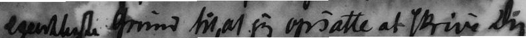egentlige Grund til, at jeg opsatte at skrive Dig
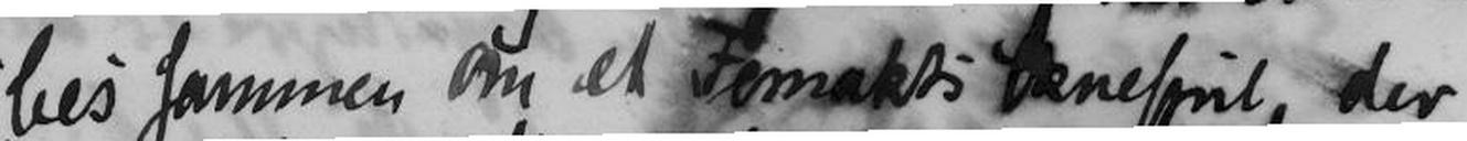bes sammen om et Femakts Skuespil, der
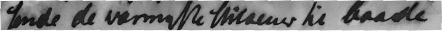sende de varmeste Hilsener til baade
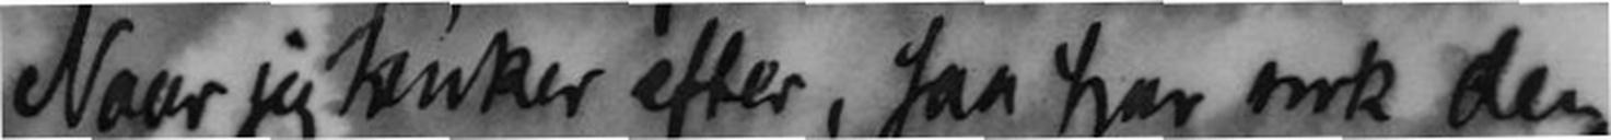Naar jeg tænker efter, saa har nok den
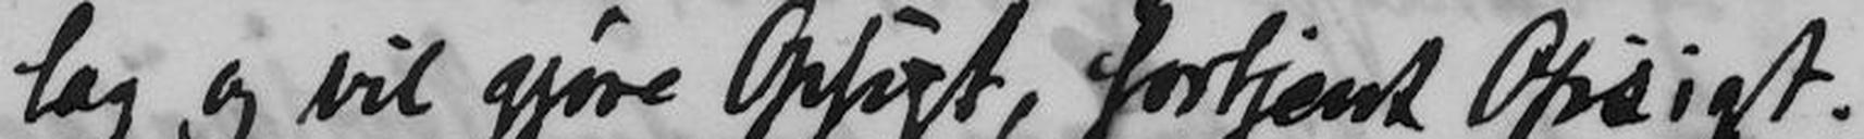lag og vil gjøre Opsigt, fortjent Opsigt.
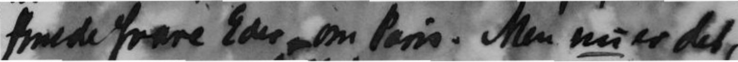skulde svare Eder om Paris. Men nu er det,
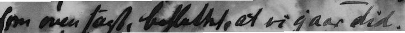som oven sagt, besluttet, at vi gaar did.
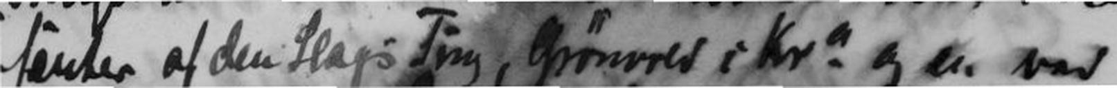santer af den Slags Ting, Grønvold i Kr? og Du ved
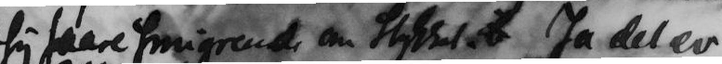sig saare smigrende om Stykket. Ja det er
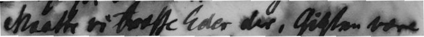Maatte vi træffe Eder der, Gigten være
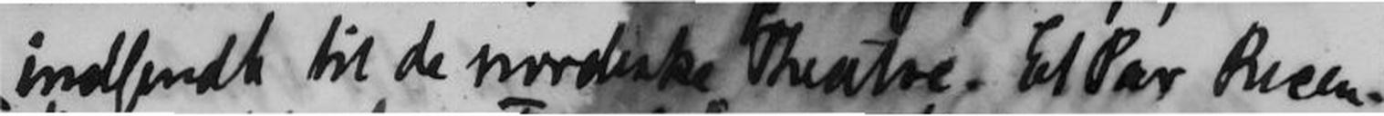indsendt til de nordiske Theatre. Et Par Recen-
